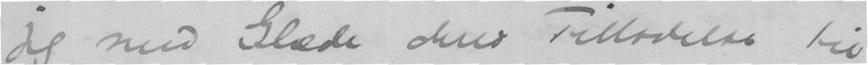jeg med Glæde deres Tilladelse til
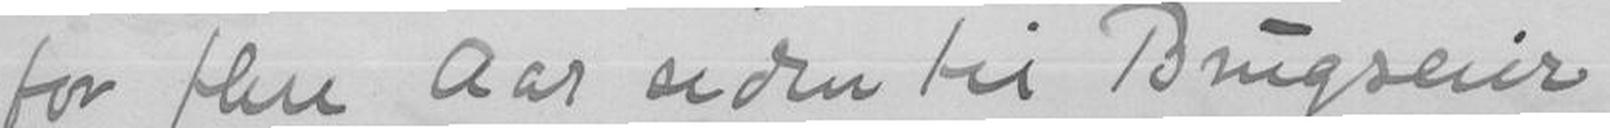for flere Aar siden til Brugseier
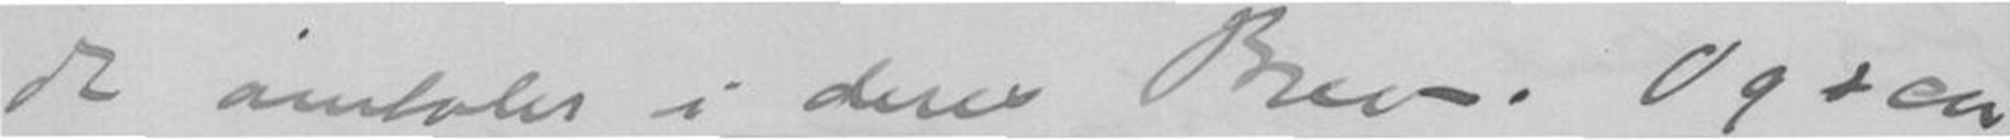de omtaler i deres Brev. Og saa
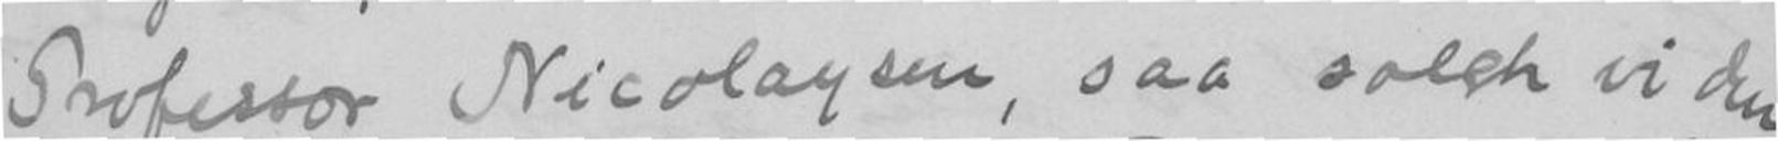Professor Nicolaysen, saa solgte vi den
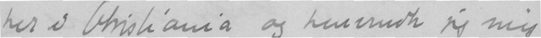her i Christiania og henvendte jeg mig
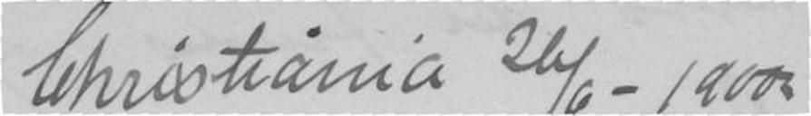Christiania 26/6 1900
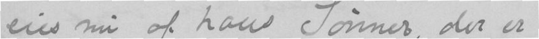eies nu af hans Sønner, der er
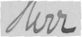Herr
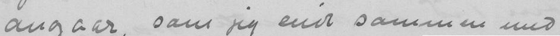angaar, som jeg eiede sammen med
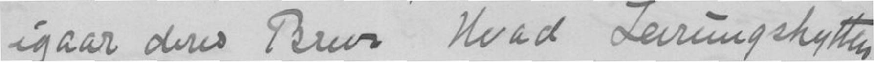igaar deres Brev. Hvad Leirungshytten
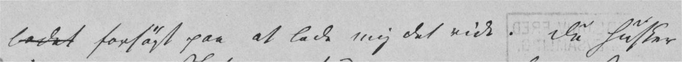ladet forsøgt paa at lade mig det vide. Du husker
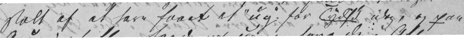stolt af at have faaet et «ug». for Tydsk idag, og paa
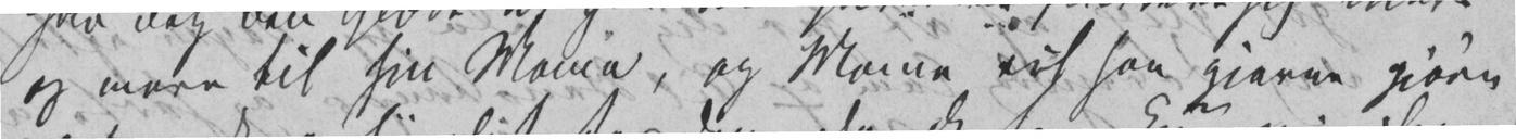og mere til sin Mama, og Mama vil saa gjerne gjøre
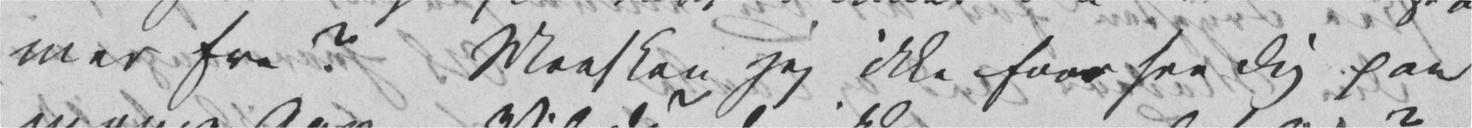mer fra? Maaskee jeg ikke faar see Dig paa
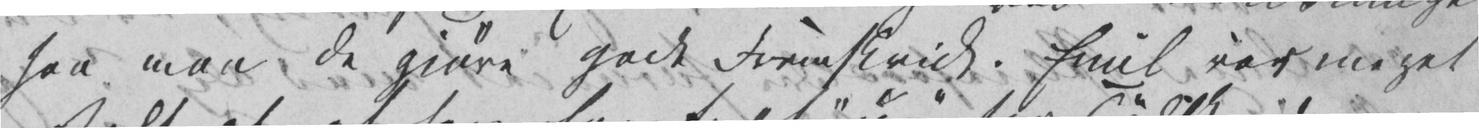saa maa de gjøre gode Fremskridt. Emil var meget
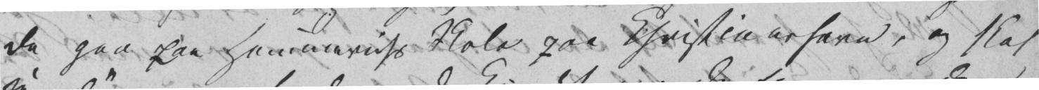De gaa paa Hammerichs Skole paa Christianshavn, og skal
